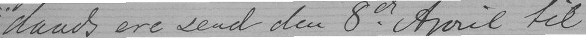dands ere send den 8de April til
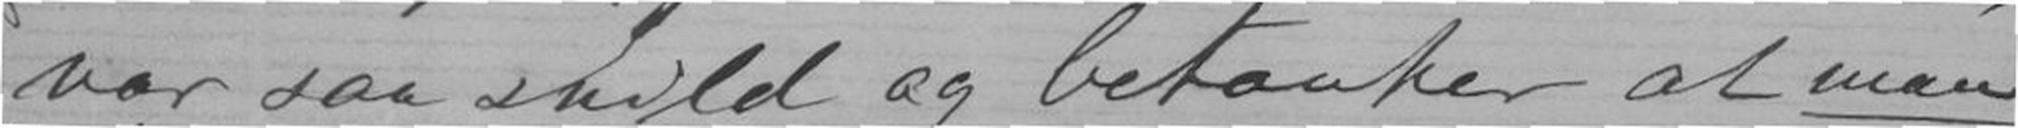vær saa snild og betænker at man
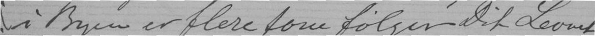i Byen er flere som følger Dit Levnet
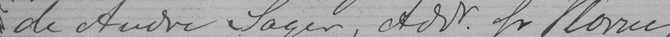de Andre Sager. Addr. hr Horne-
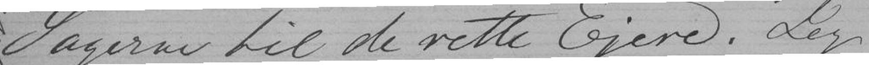Sagerne til de rette Ejere. Jeg
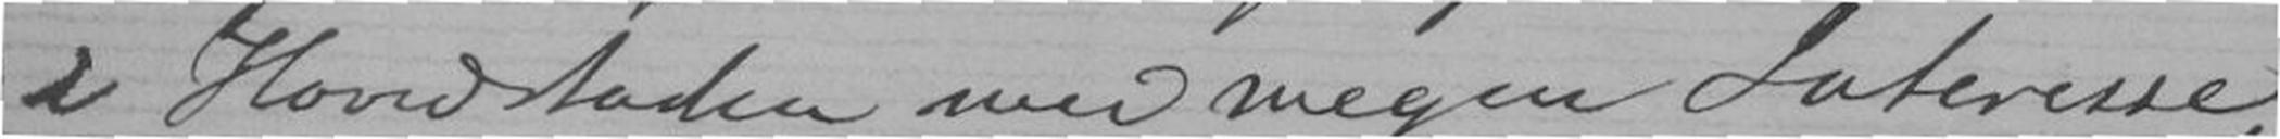i Hovedstaden med megen Interesse,
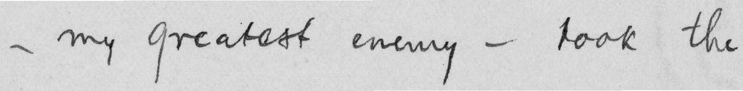- my greatest enemy - took the
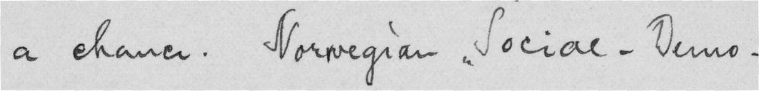a chance. Norwegian "Social-Demo-
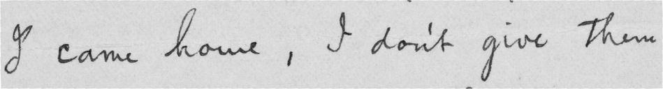I came home, I don't give them
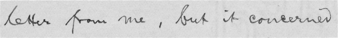letter from me, but it concerned
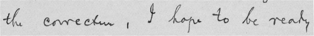the correctur, I hope to be ready
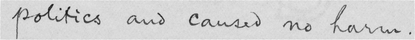politics and caused no harm.
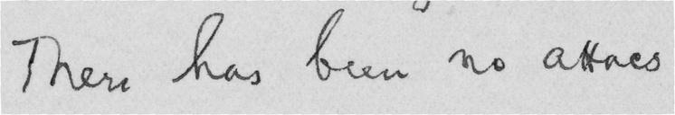There has been no attacs
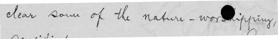clear some of the nature-worshipping,
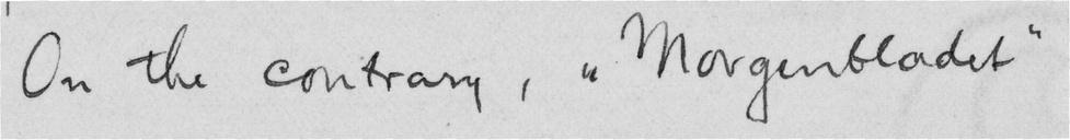On the contrary, "Morgenbladet"
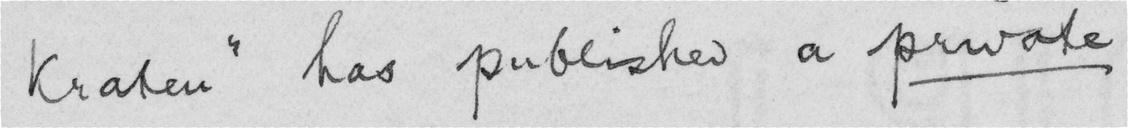kraten" has published a private
